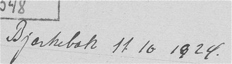Bjerkebæk 11.10. 1924.
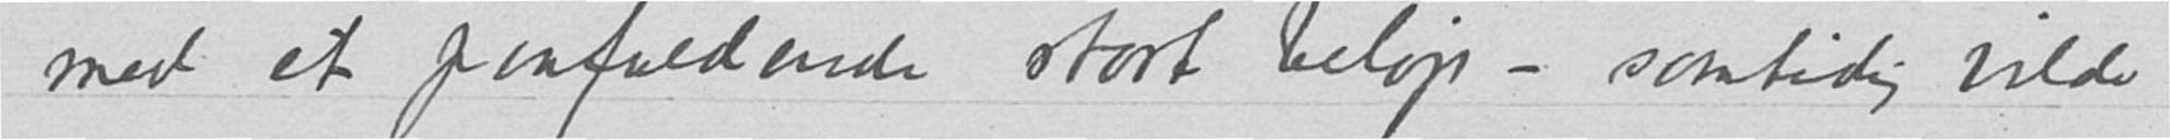med et paafaldende stort beløp - samtidig vilde
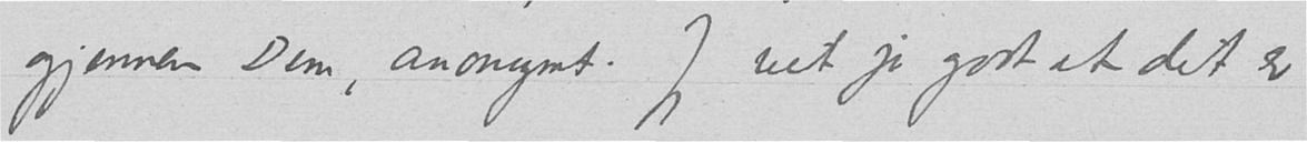gjennem Dem, anonymt. Jeg vet jo godt at det er
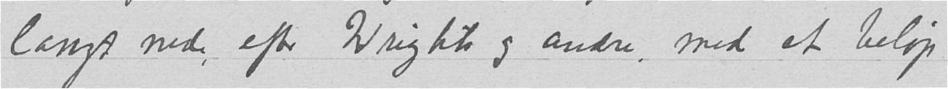langt nede, efter Wrights og andre, med et beløp
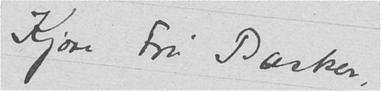Kjære Fru Backer,
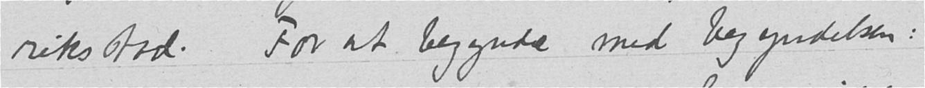riksstad. For at begynde med begyndelsen:
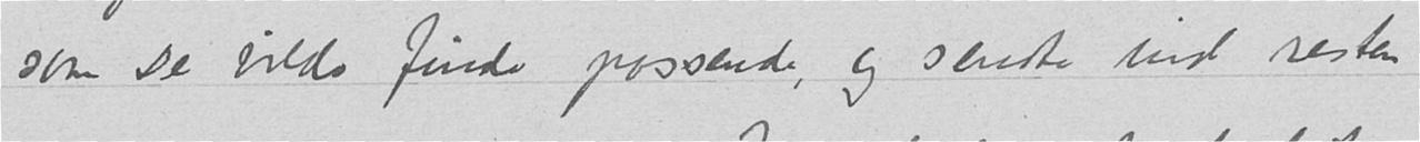som De vilde finde passende, og sendte ind resten
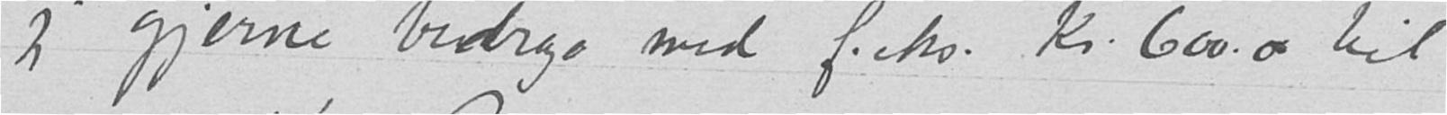jeg gjerne bidrage med f.eks. Kr. 600.00 til
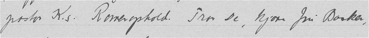pastor K.s. Romerophold. Tror De, kjære fru Backer,
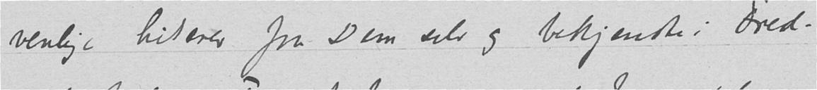venlige hilsener fra Dem selv og bekjendte i Fred-
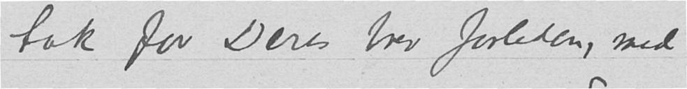tak for Deres brev forleden, med
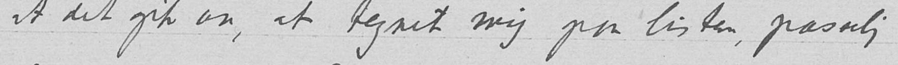at det gik an, at tegnet mig paa listen, passelig
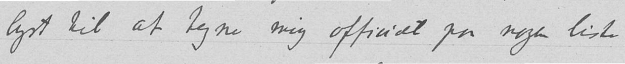lyst til at tegne mig officielt paa nogen liste
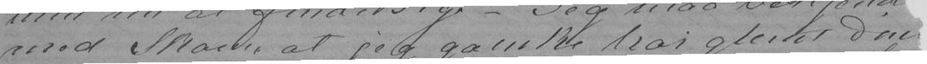med Skam at jeg ganske har glemt Din
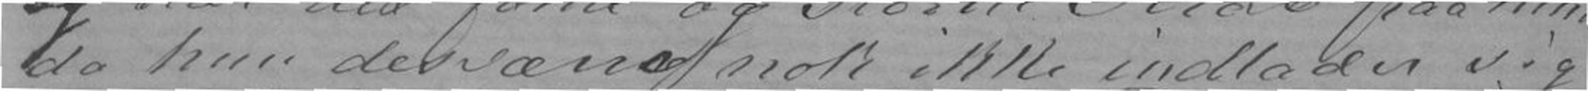da hun desværre nok ikke indlader sig
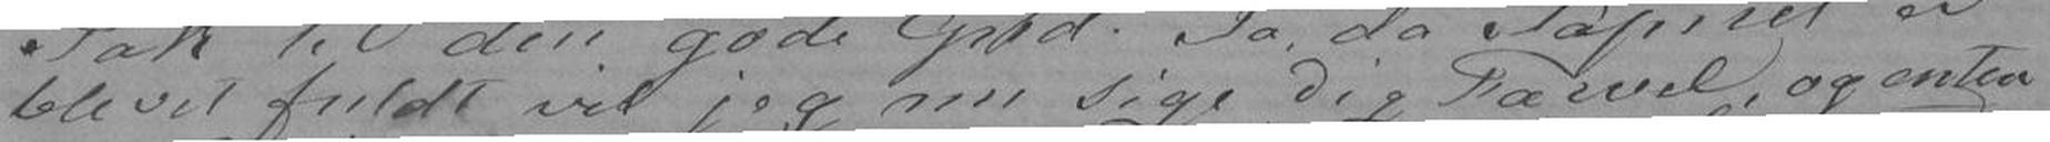blevet fuldt vil jeg nu sige Dig Farvel, og enten
